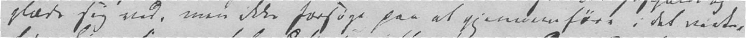glæde sig ved, men ikke forsøge paa at gjennemføre i det virke-
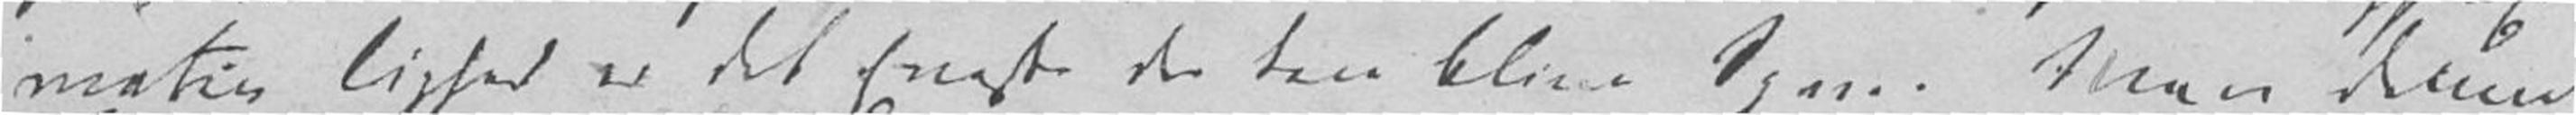mativ Lighed er det Eneste der kan blive Spm. Men denne
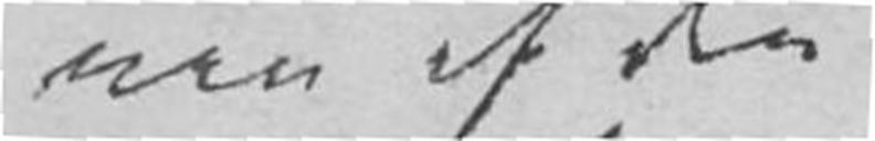nen af den
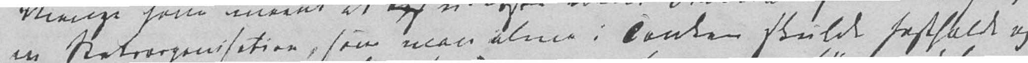en Statsorganisation, som man alene i Tanken skulde fastholde og
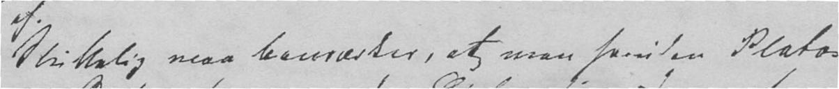Sluttelig maa bemærkes, at man foruden Platos
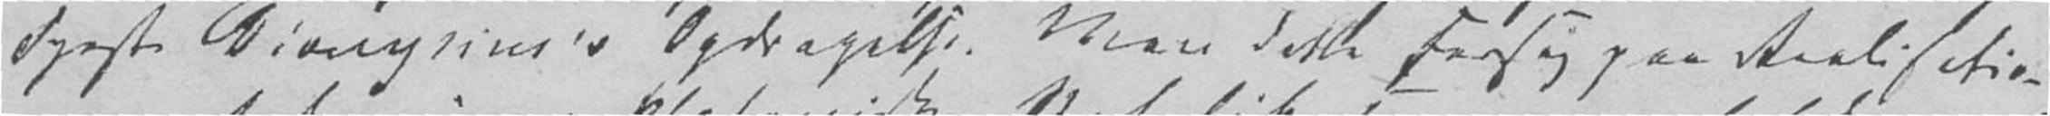Fyrste Dionysius's Opdragelse. Men dette Forsøg paa Realisatio-
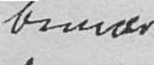bemær-
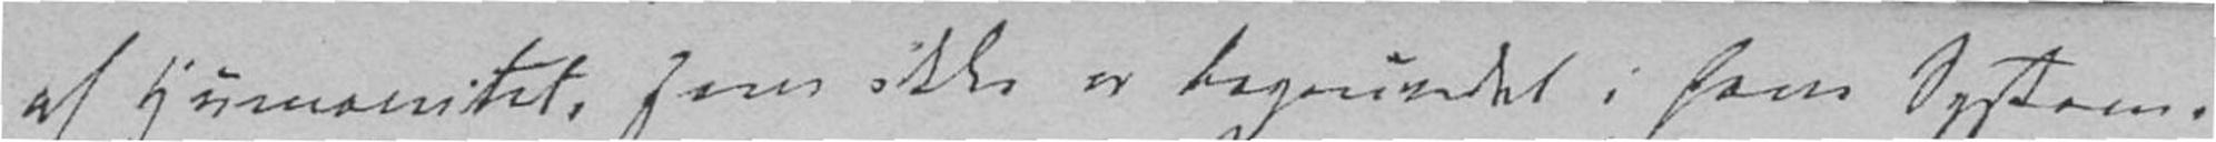af Humanitet, som ikke er begrundet i hans System.
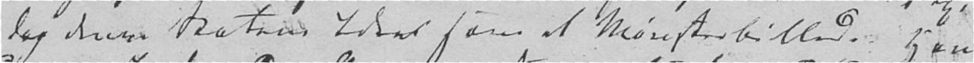dog denne Statens Idee som et Mønsterbillede. Han
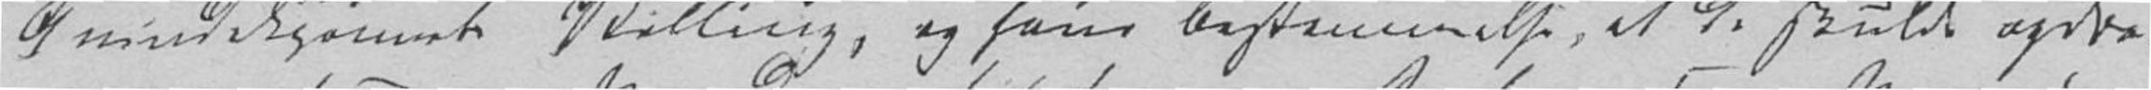Qvindekjønnets Stilling, og hans Bestemmelse, at de skulde opdra-
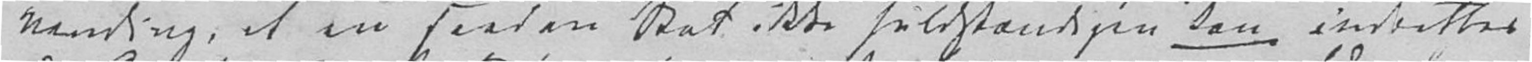vending, at en saadan Stat ikke fuldstændigen kan indrettes.
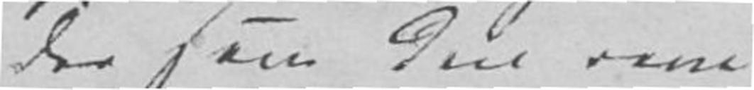der som den rene
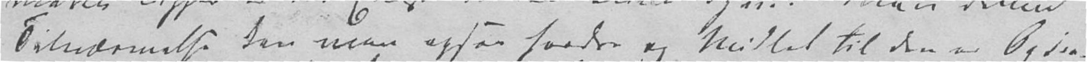Tilnærmelse kan man ogsaa fordre og Midlet til den er Opdra-
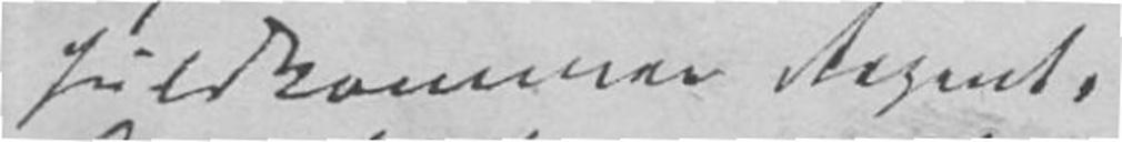fuldkommen Regent,
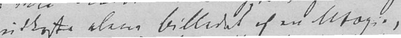udkaste alene Billedet af en Utopie,
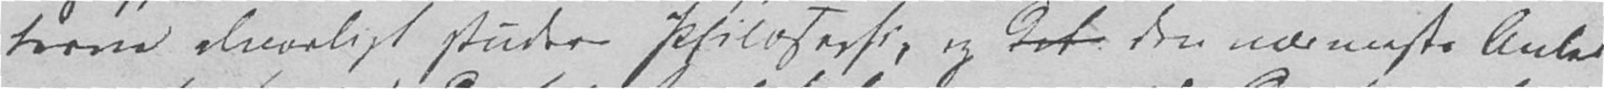terne alvorligt studere Philosophi, og det den nærmeste Anled-
